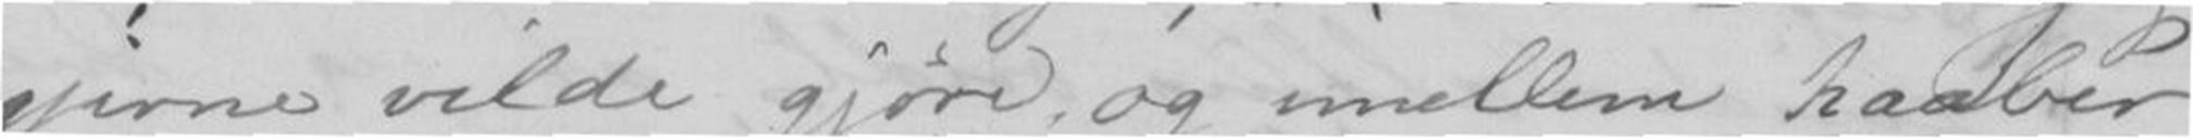gjerne vilde gjøre og imellem haaber
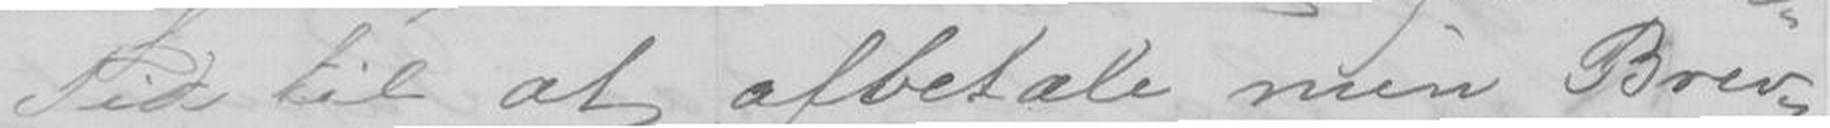Tid til at afbetale min Brev-
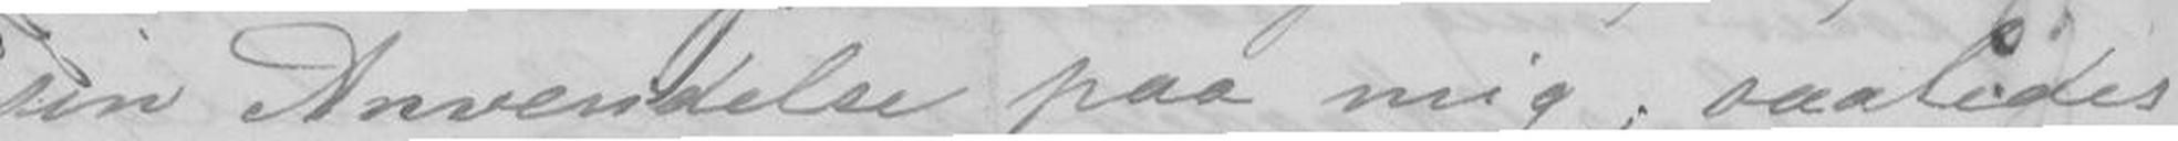sin Anvendelse paa mig; saaledes
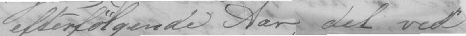ge efterfølgende Aar, det ved
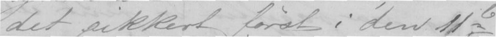det sikkert først i den 11te
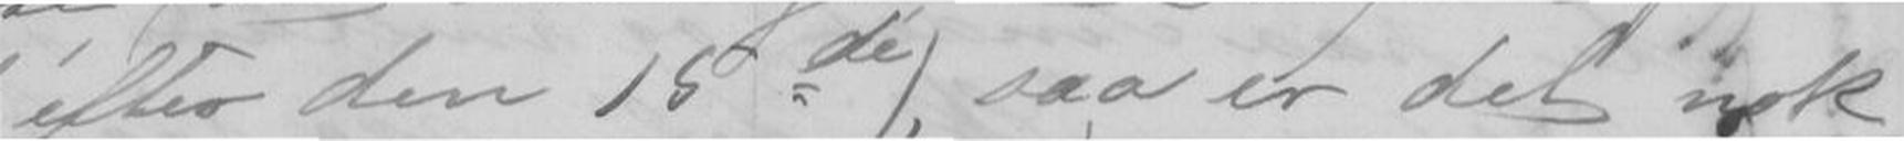efter den 15de), saa er det nok
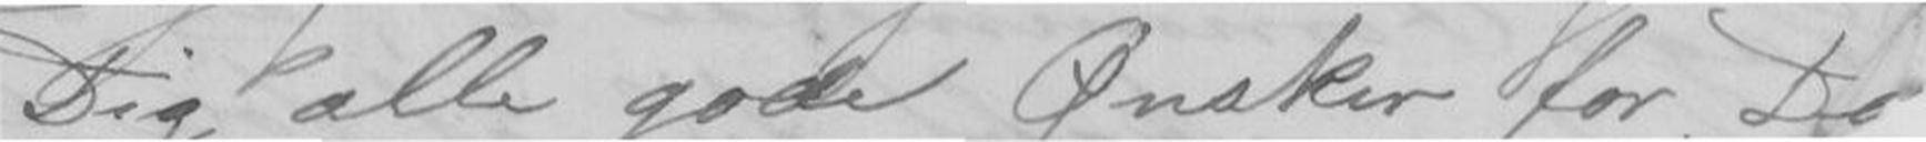de Dig alle gode Ønsker for D
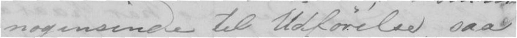nogensinde til Udførelse, saa
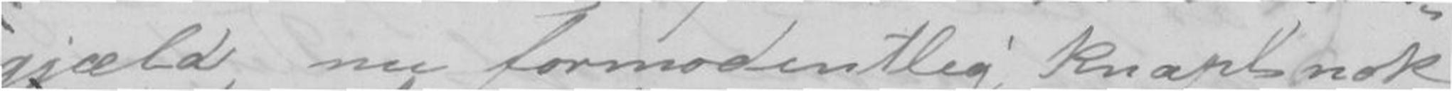gjæld, nu formodentlig knapt nok
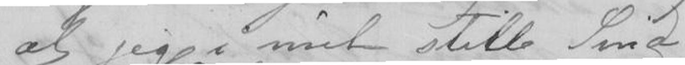for at sige i mit stille Sind
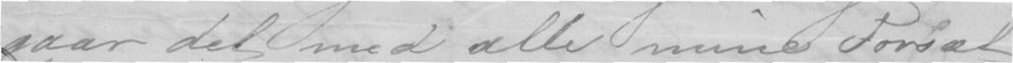gaar det med alle mine Forsæt-
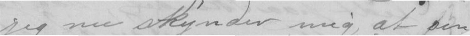jeg nu skynder mig at sen-
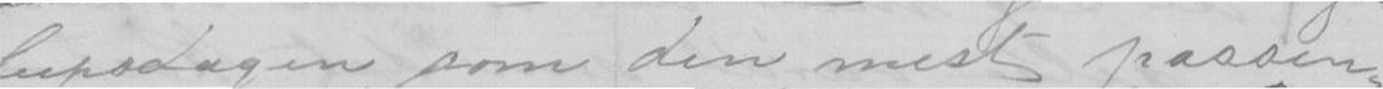lupsdagen som den mest passen-
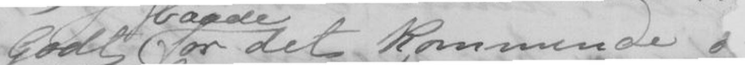Godt for det kommende
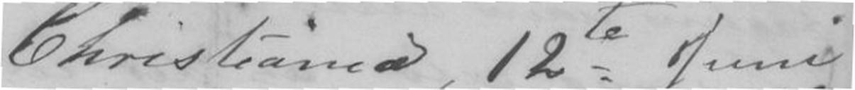Christinia, 12te Juni
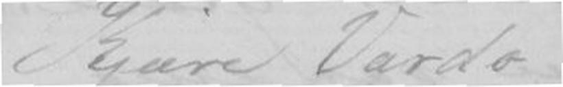Kjære Vardo!
